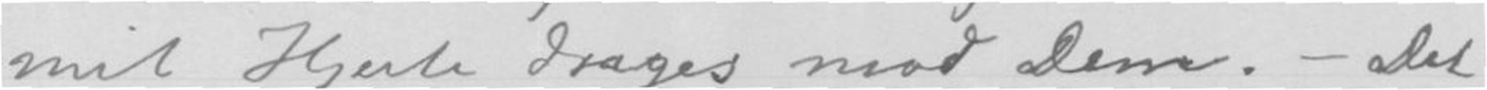mit Hjerte drages mod Dem. - Det
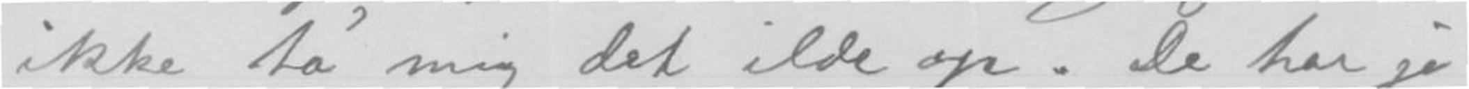ikke ta' mig det ilde op. De har jo
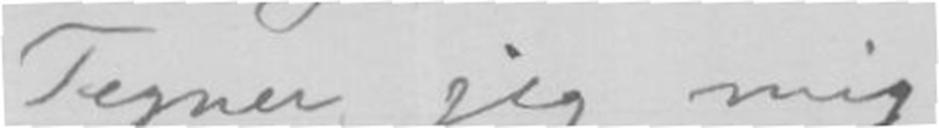Tegner jeg mig
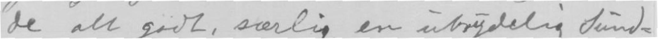de alt godt, særlig en ubrydelig Sund-
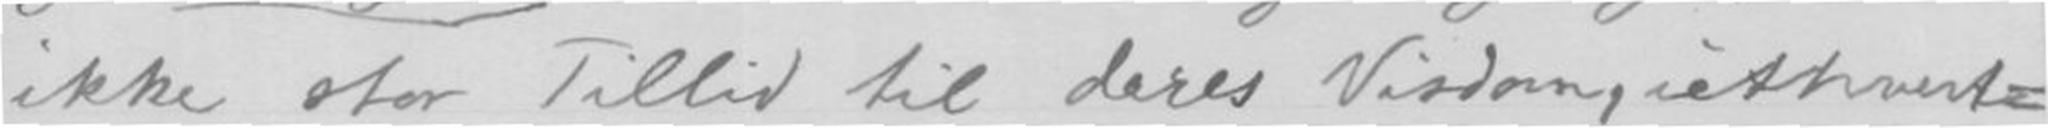ikke stor Tillid til deres Visdom, iethvert-
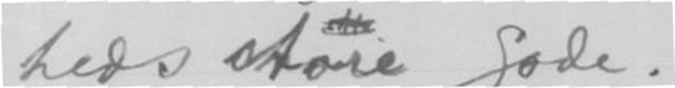heds store Gode.
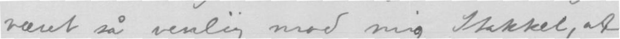været så venlig mod mig Stakkel, at
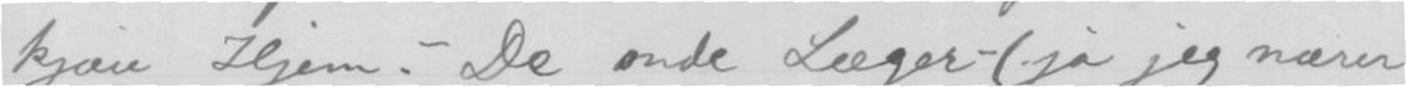kjære Hjem. - Det onde Læger - (ja jeg nærer
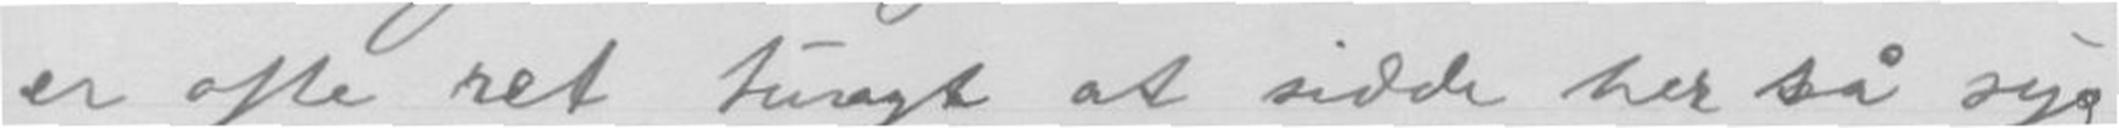er ofte ret tungt at sidde her så syg
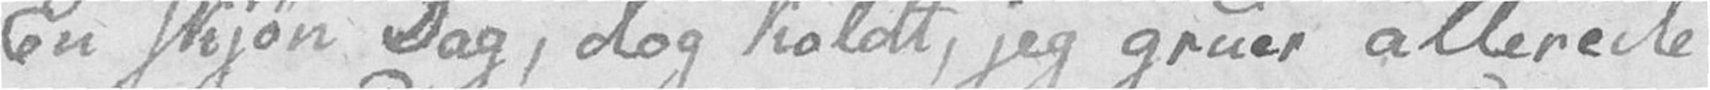En skjøn Dag, dog koldt, jeg gruer allerede
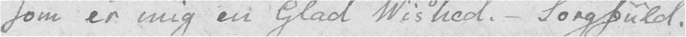som er mig en Glad Vished. – Sorgfuld.
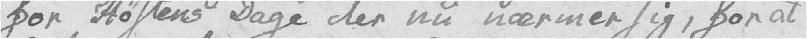for Høstens Dage der nu nærmer sig, for at
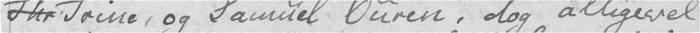Thr Trine, og Samuel Ouren, dog alligevel
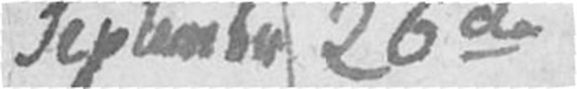September 26de
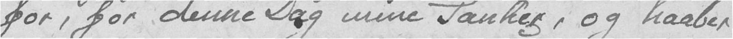for, for denne Dag mine Tanker, og haaber
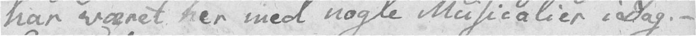har været her med nogle Musicalier i Dag. –
